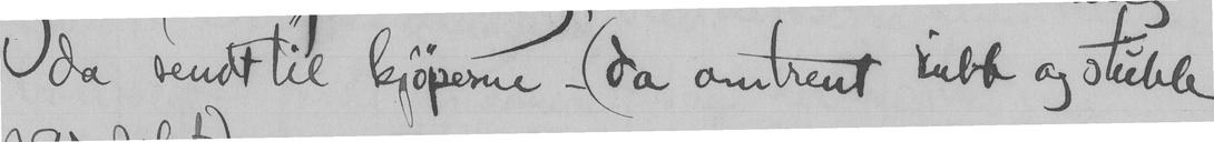da sendt til kjøperne - (da omtrent rubb og stuble
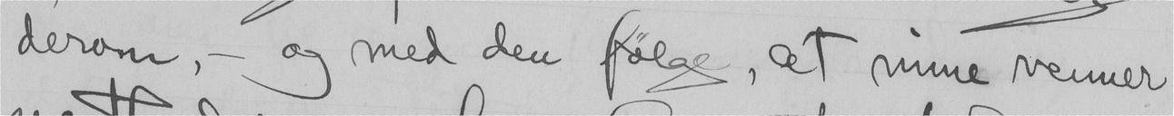derom, - og med den følge, at mine venner
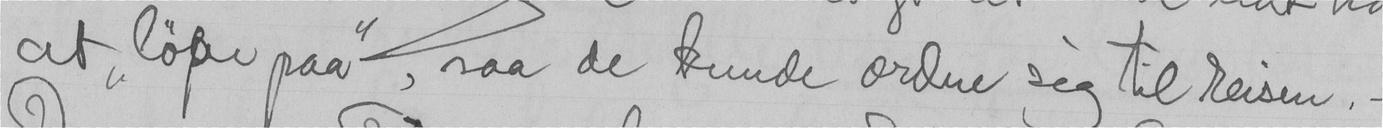at "løpe" paa" - saa de kunde ordne sig til reisen. -
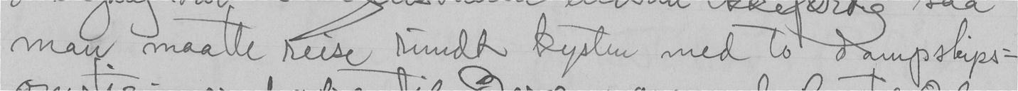man maatte reise rundt kysten med tot dampskips-
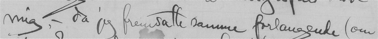mig, - da jeg fremsatte samme forlangende (om
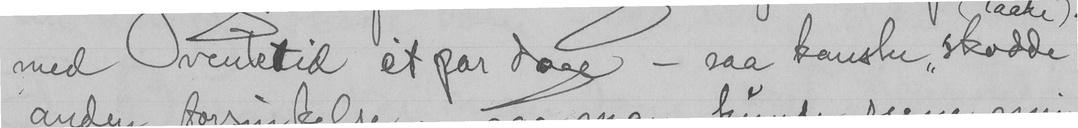med ventetid et par dage - saa kanske "skodde"
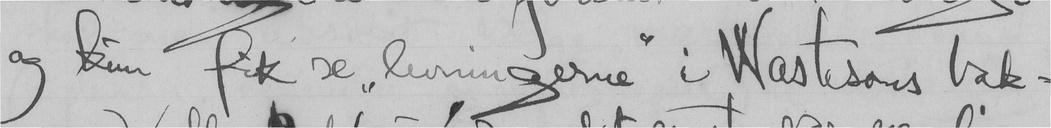og kun fik se "levningerne" i Wastesons bak-
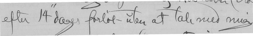efter 14 dages forløb - uten at tale med mig
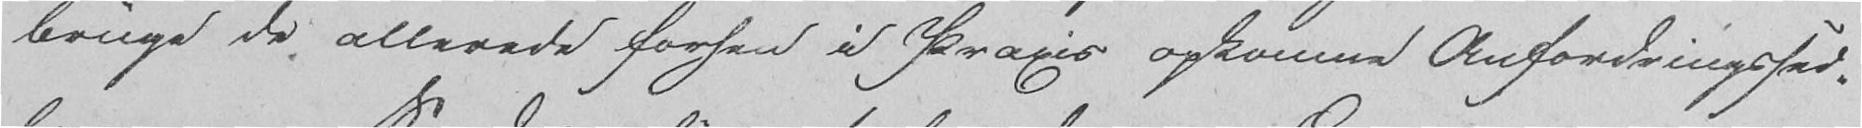bruge de allerede forhen i Praxis opkomne Anfordringssed-
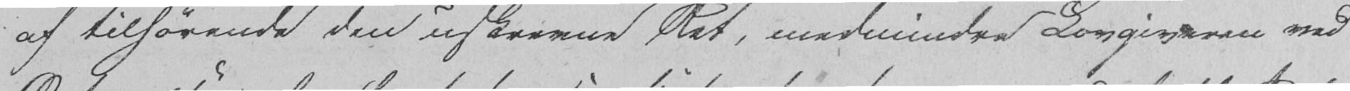af tilhørende den uskrevne Ret, medmindre Lovgiveren ved
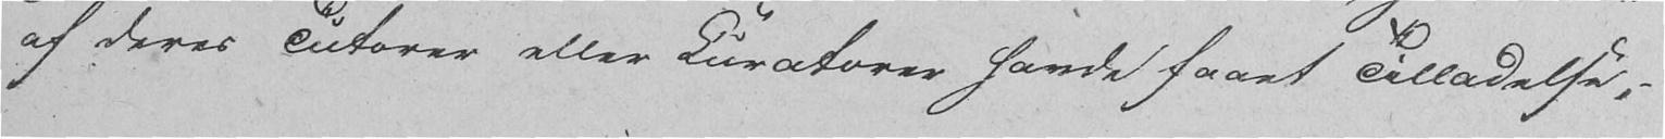af Deres Tutorer eller Kuratorer havde faaet Tilladelse, -
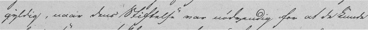gyldig, naar dens Stiftelse var nødvendig for at de kunde
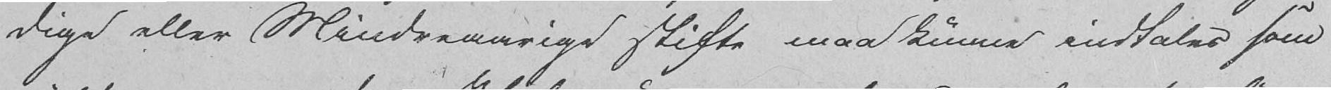dige eller Mindreaarige stifte maa kunne indtales som
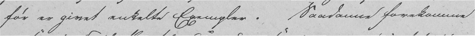før er givet enkelte Exempler. Saadanne forekomme
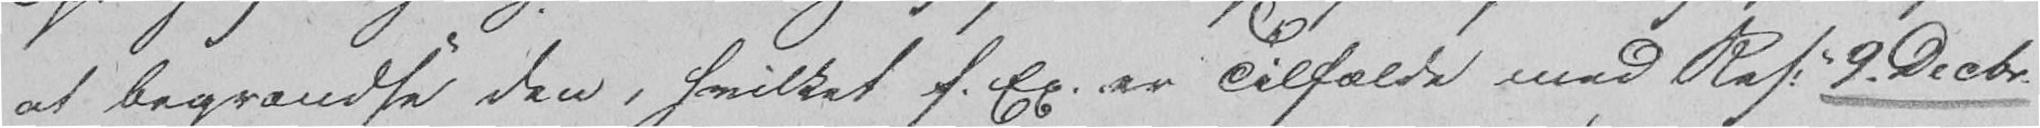at begrændse den, hvilket f. Ex. er Tilfælde med Res. 9. Decbr.
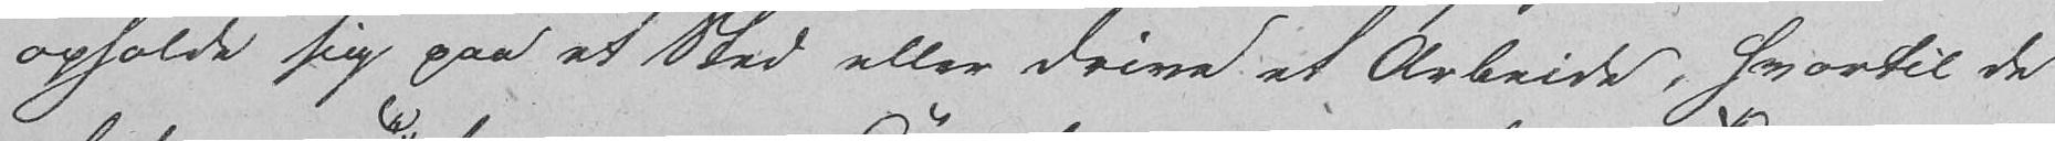opholde sig paa et Sted eller drive et Arbeide, hvortil de
